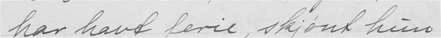har havt ferie, skjønt hun
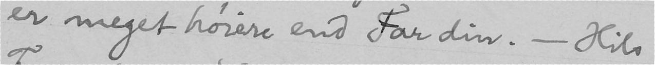er meget høiere end Far din. - Hils
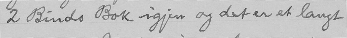2 Binds Bok igjen og det er et langt
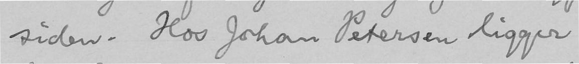siden. Hos Johan Petersen ligger
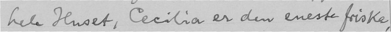hele Huset, Cecilia er den eneste friske,
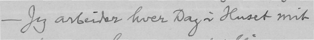- Jeg arbeider hver Dag i Huset mit
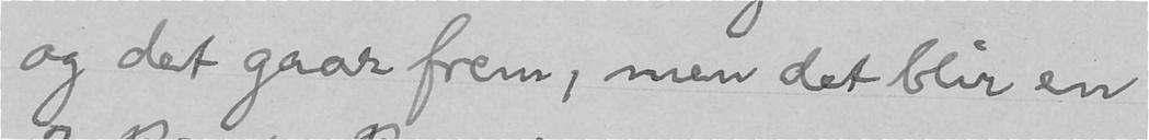og det gaar frem, men det blir en
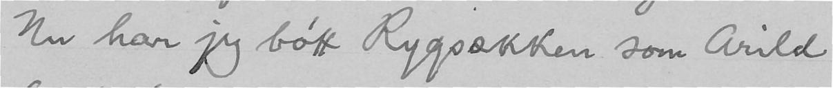Nu har jeg bøtt Rygsækken som Arild
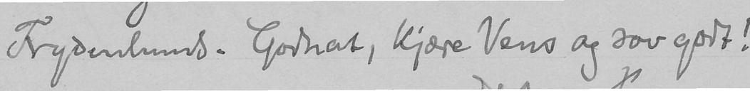Frydenlund. Godnat, kjære Vens og sov godt!
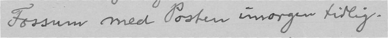Fossum med Posten imorgen tidlig.
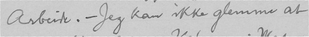Arbeide. - Jeg kan ikke glemme at
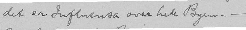det er Influensa over hele Byen. -
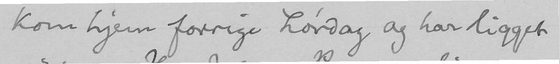kom hjem forrige Lørdag og har ligget
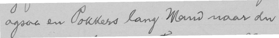ogsaa en Pokkers lang Mand naar du
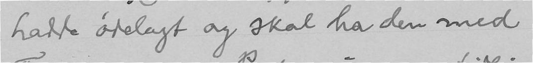hadde ødelagt og skal ha den med
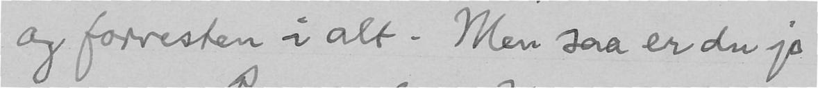og forresten i alt. Men saa er du jo
